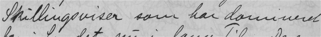Skillingsviser som har domineret
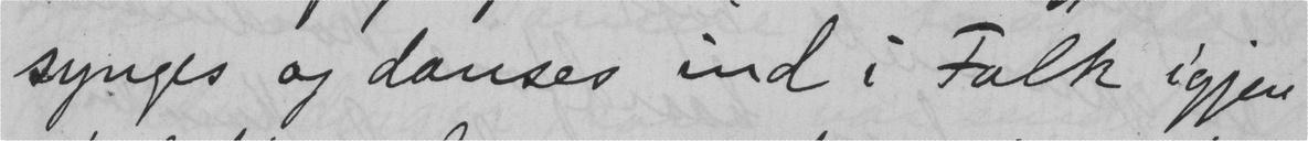synges og danses ind i Folk igjen
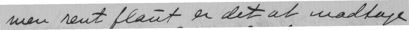men rent flaut er det at modtage
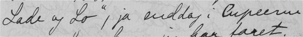Lade og Lo", ja enddog i Cupeerne
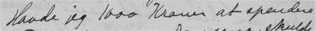Havde jeg 1000 Kroner at spendere
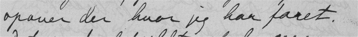opover der hvor jeg har faret.
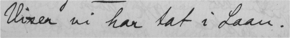Viser vi har tat i Laan.
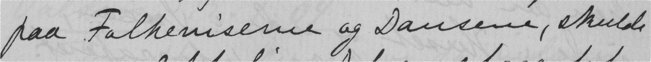paa Folkeviserne og Danserne, skulde
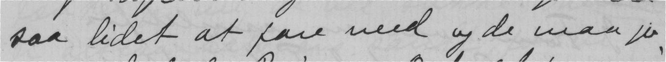saa lidet at fare med og de maa jo
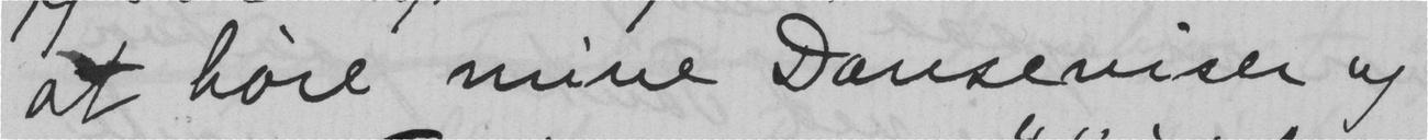at høre mine Danseviser og
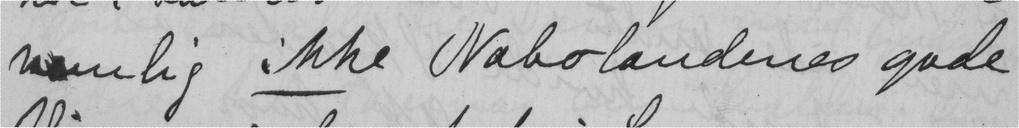nemlig ikke Nabolandenes gode
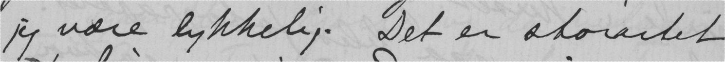jeg være lykkelig. Det er storartet
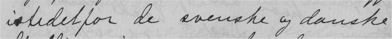istedetfor de svenske og danske
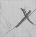x
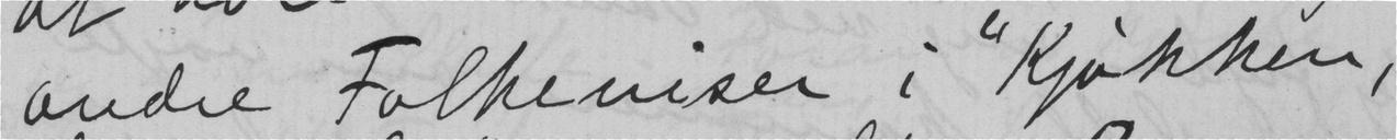andre Folkeviser i "Kjøkken,
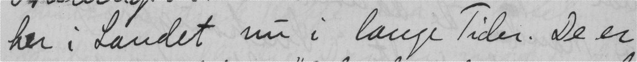her i Landet nu i lange Tider. De er
